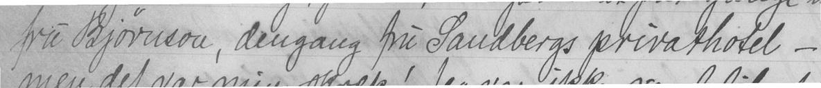fru Bjørnson, dengang fru Sandbergs privathadel -
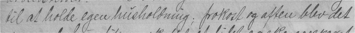til at holde egen husholdning; frokost og aften blev det
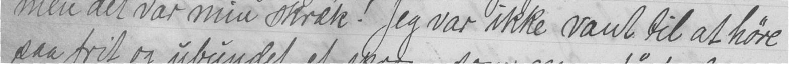men det var min skræk! Jeg var ikke vant til at høre
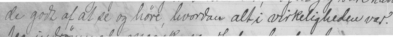de godt af at se og høre, hvordan alt i virkeligheden var.
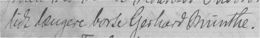lidt længere borte Gerhard Munthe.
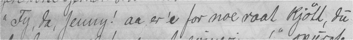"Fy, da, Jenny! aa er'e for noe raat kjøtt, du
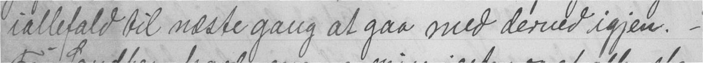iallefald til næste gang at gaa med derned igjen. -
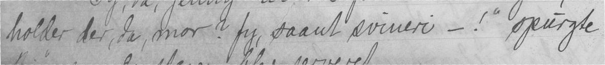holder der, da, mor? fy, saant svineri - !" spurgte
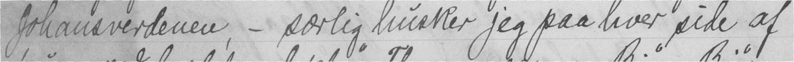Johansverdenen, - særlig husker jeg paa hver side af
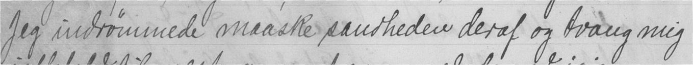Jeg indrømmede maaske sandheden deraf og tvang mig
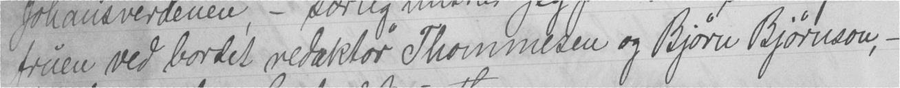fruen ved bordet redaktør Thommesen og Bjørn Bjørnson, -
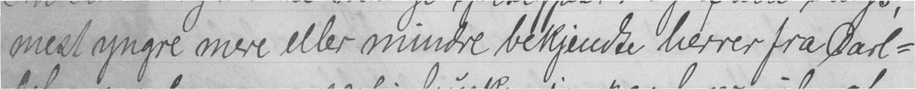mest yngre mere eller mindre bekjendte herrer fra Carl-
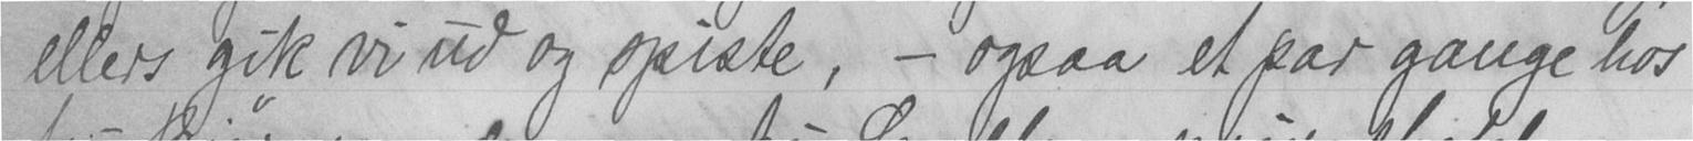ellers gik vi ud og spiste, - ogsaa et par gange hos
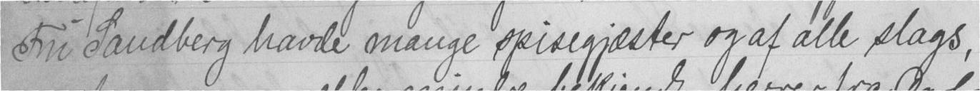Fru Sandberg havde mange spisegjæster og af alle slags,
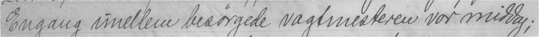Engang imellem besørgede vagtmesteren vor middag;
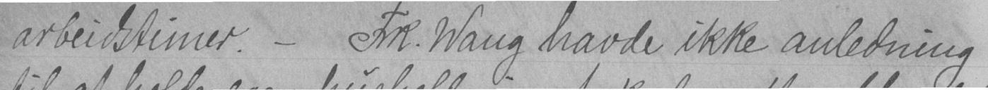arbeidstimer. - Frk. Wang havde ikke anledning
